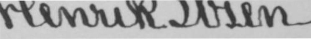Henrik Ibsen.
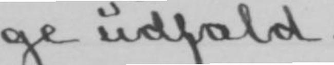ge udfald.
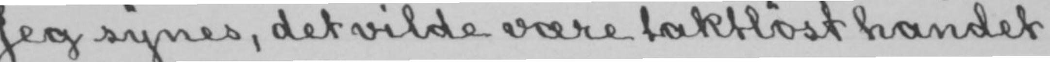Jeg synes, det vilde være taktløst handet
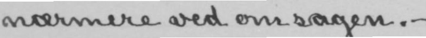nærmere ved om sagen.-
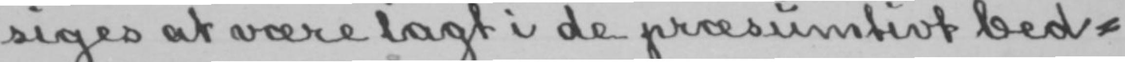siges at være lagt i de præsumtivt bed-
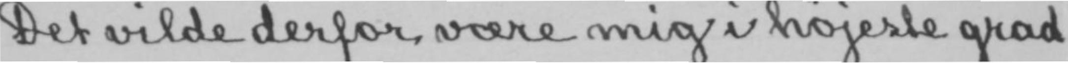Det vilde derfor være mig i højeste grad
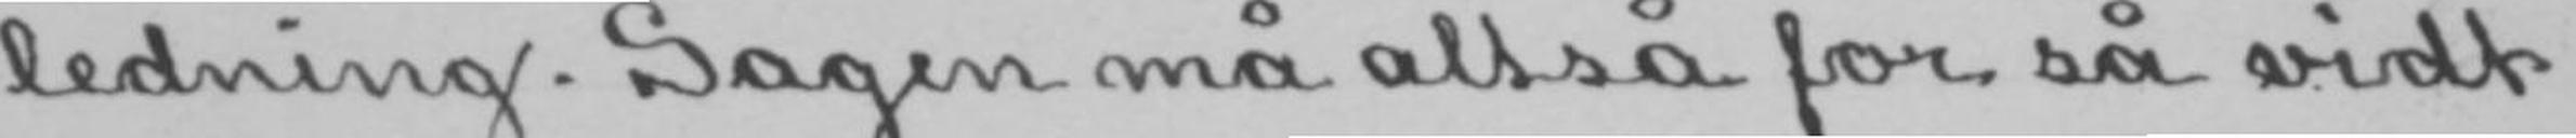ledning. Sagen må altså for så vidt
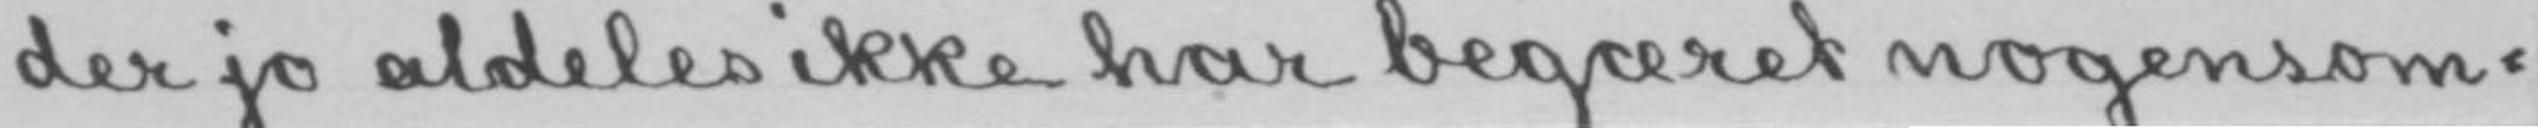der jo aldeles ikke har begæret nogensom-
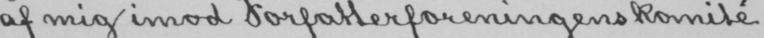af mig imod Forfatterforeningens komité
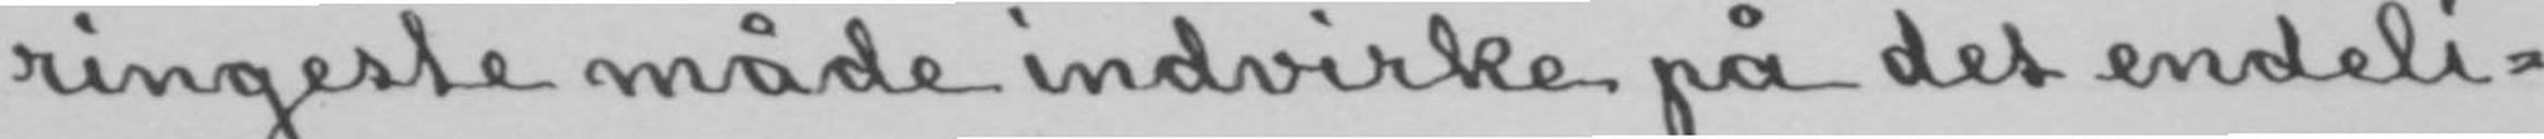ringeste måde indvirke på det endeli-
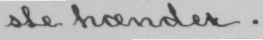ste hænder.
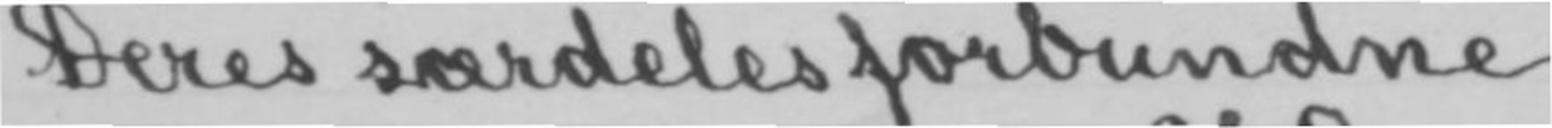Deres særdeles forbundne
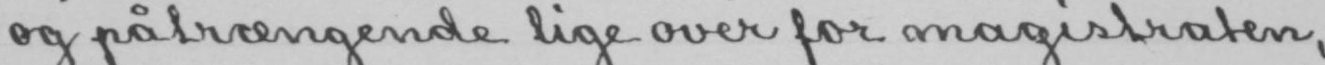og påtrængende lige over for magistraten,
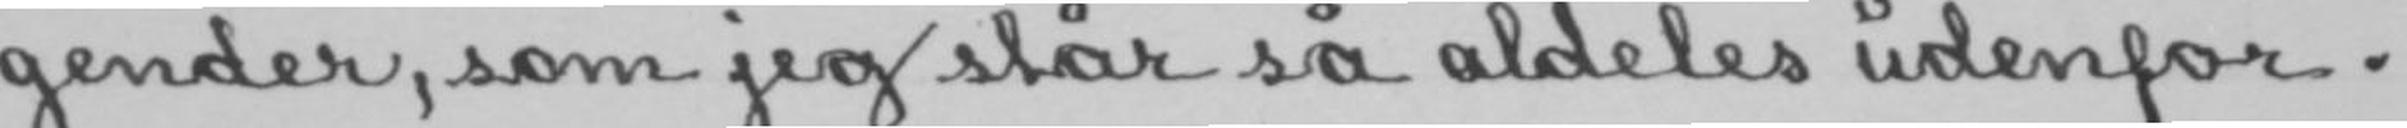gender, som jeg står så aldeles udenfor.
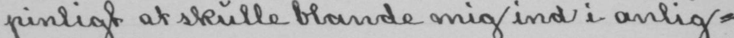pinligt at skulle blande mig ind i anlig-
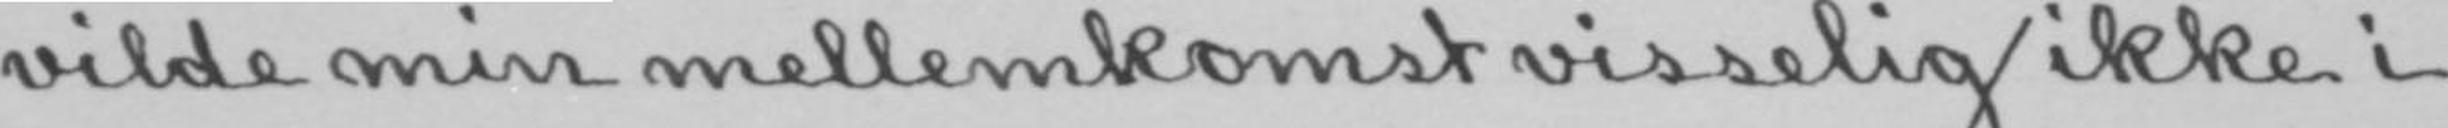vilde min mellemkomst visselig ikke i
